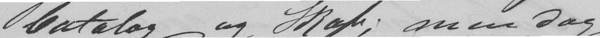Catalog og Skap; men dog
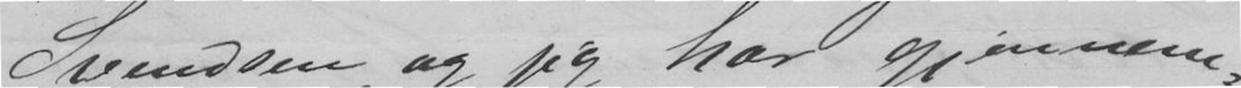Svendsen og jeg har gjennem-
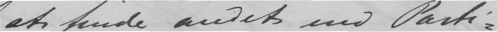at finde andet end Parti-
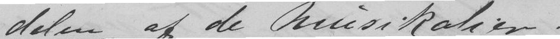delen af de Musikalier;
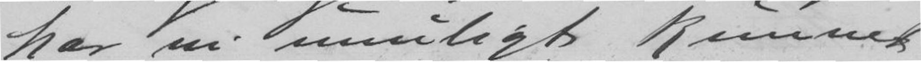har vi umuligt kunnet
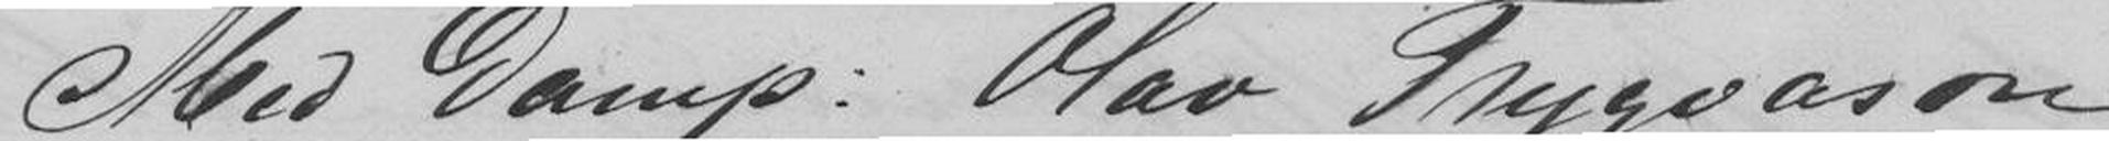Med Damp: Olav Trygvason
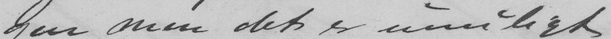gen men det er umuligt
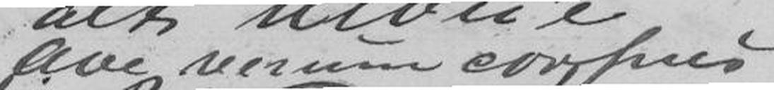Ave verum corpus
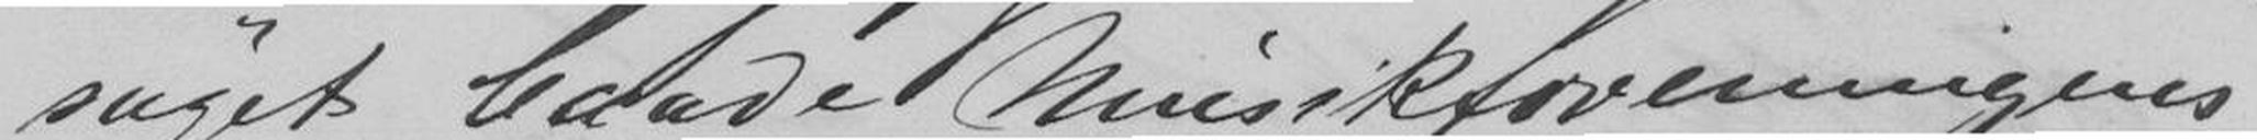søget baade Musikforeningens
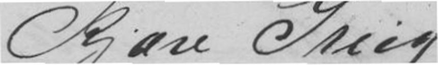Kjære Grieg
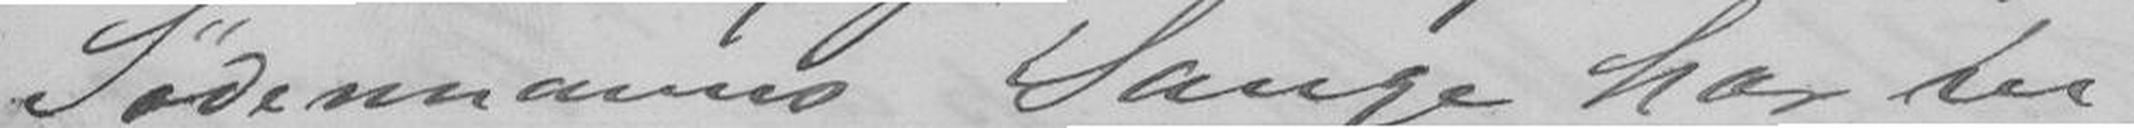Sødermanns Sange har vi
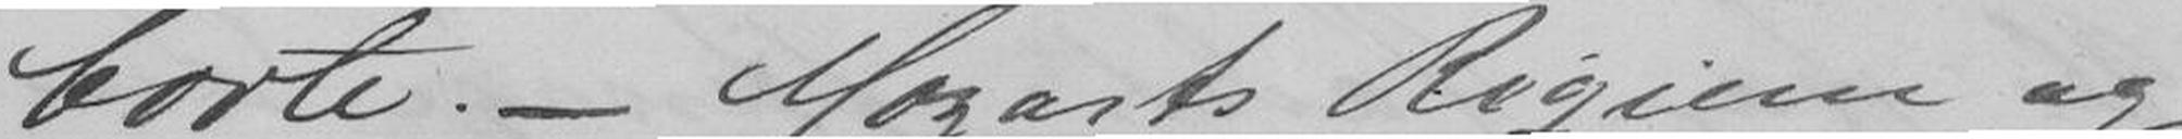borte. - Mozarts Reqiem og
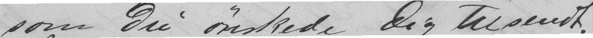som Du ønskede Dig tilsendt.
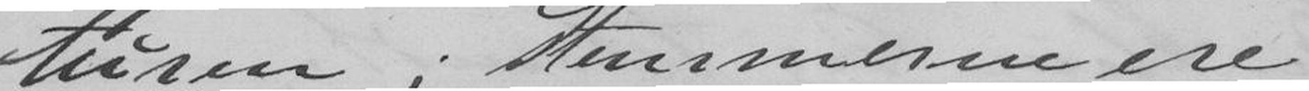turen; Stemmerne ere
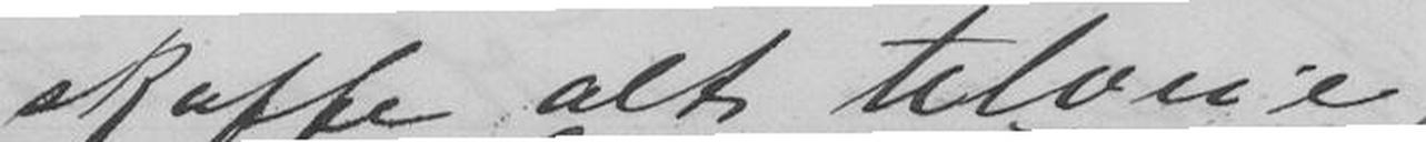skaffe alt tilveie
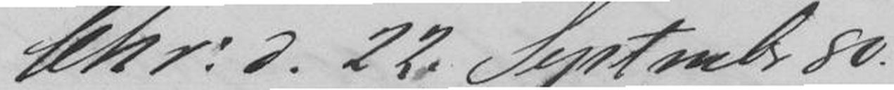Chr: d. 22 Septmbr 80.
